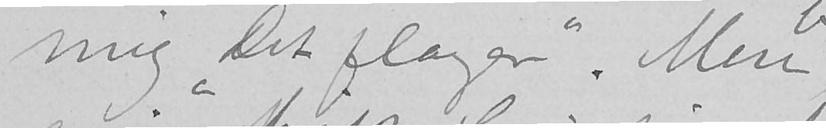mig "Det flager". Men
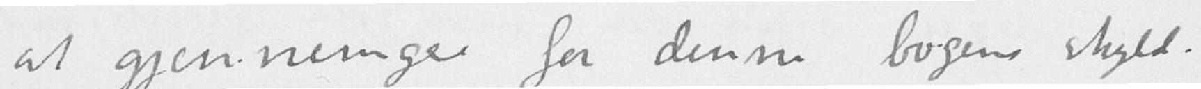at gjennemgaa for denne bogens skyld.
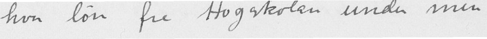hver løn fra Høgskolan under min
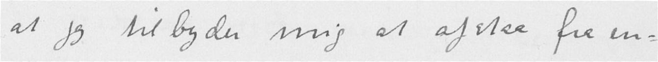at jeg tilbyder mig at afstaa fra en-
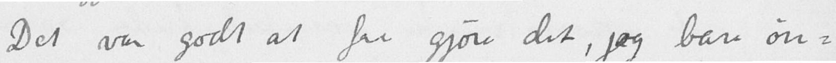Det var godt at faa gjøre det, jeg bare øn-
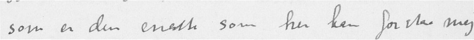som er den eneste som her kan forstaa mig.
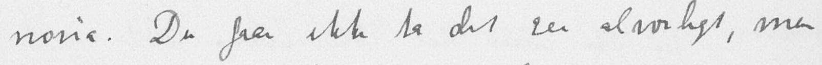noria. Du faar ikke ta det saa alvorligt, men
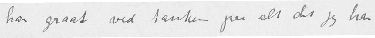har graat ved tanken paa alt det jeg har
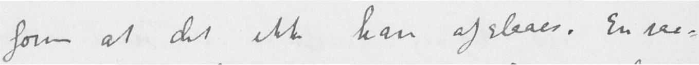form at det ikke kan afslaaes. En saa-
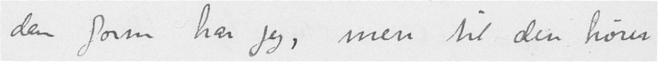dan form har jeg, men til den hører
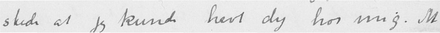skede at jeg kunde havt dig hos mig. At
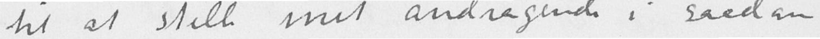til at stille mit andragende i saadan
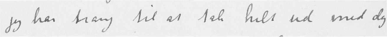jeg har trang til at tale helt ud med dig
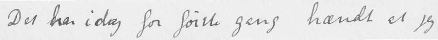Det har i dag for første gang hændt at jeg
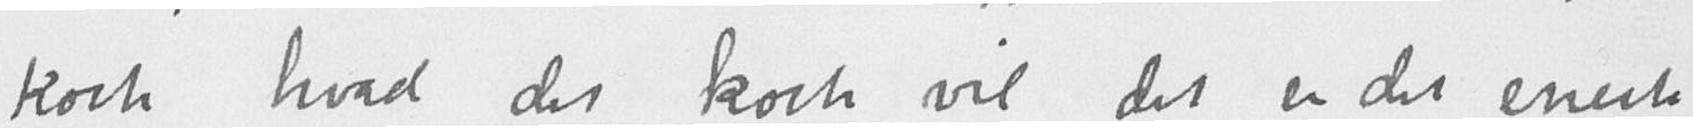koste hvad det koste vil det er det eneste
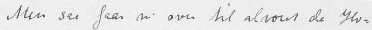Men saa faar vi over til alvoret da Ho-
